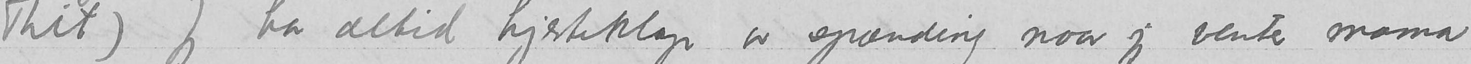Jeg har altid hjerteklap av spænding naar jeg venter mama
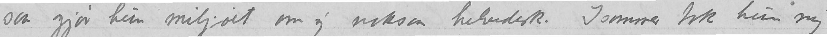saa gjør hun miljøet om sig noksaaå helvedesk. I sommer tok hun mig
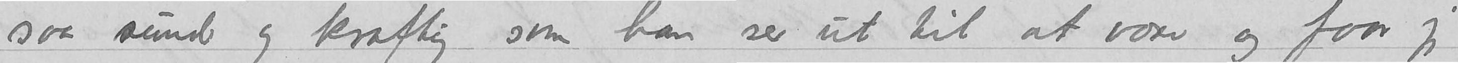saa sund og kraftig som han ser ut til at være og faar jeg
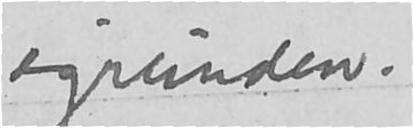igrunden
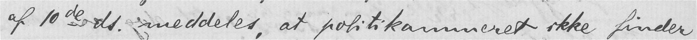af 10de ds. meddeles, at politikammeret ikke finder
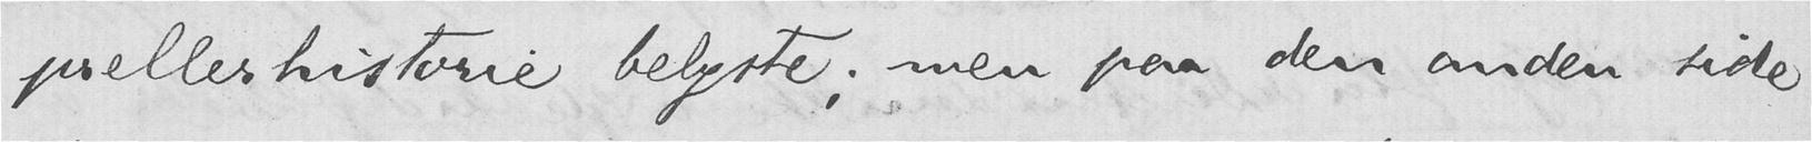prellerhistorie belyste; men paa den anden side
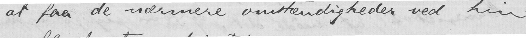at faa de nærmere omstændigheder ved hin
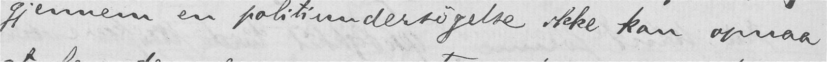gjennem en politiundersøgelse ikke kan opnaa
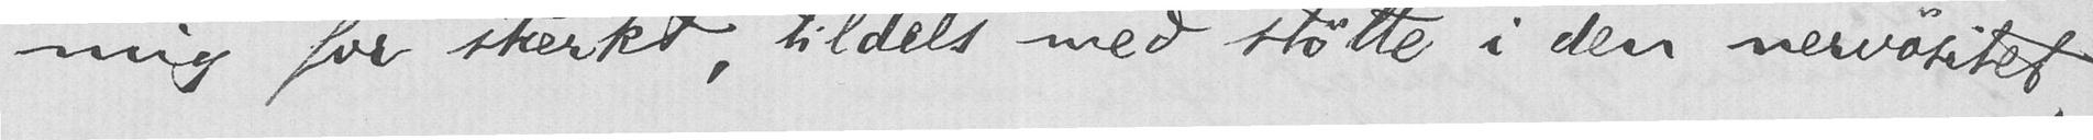mig for stærkt, tildels med støtte i den nervøsitet,
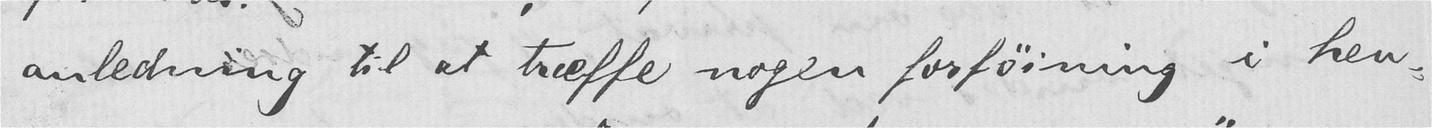anledning til at træffe nogen forføining i hen-
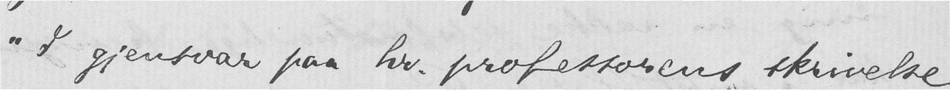"I gjensvar paa hr. professorens skrivelse
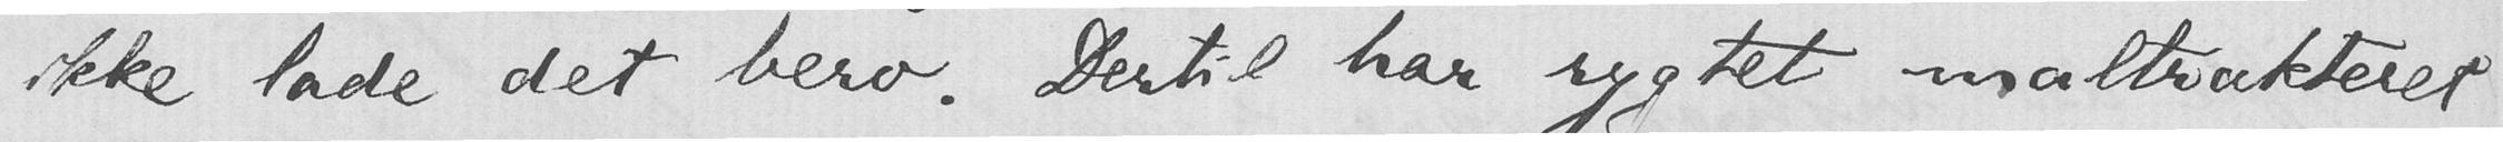ikke lade det bero. Dertil har rygtet maltrakteret
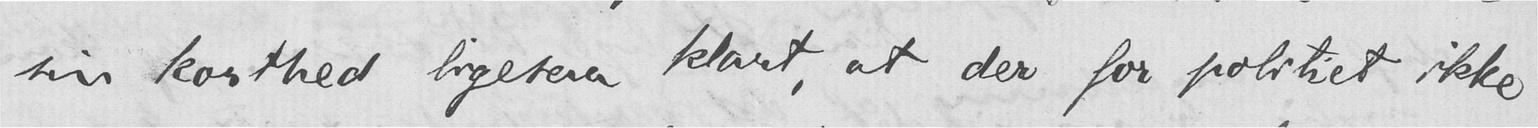sin korthed ligesaa klart, at der for politiet ikke
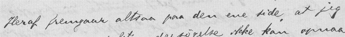Heraf fremgaar altsaa paa den ene side, at jeg
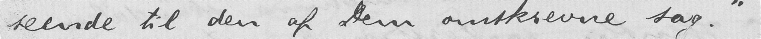seende til den af Dem omskrevne sag."
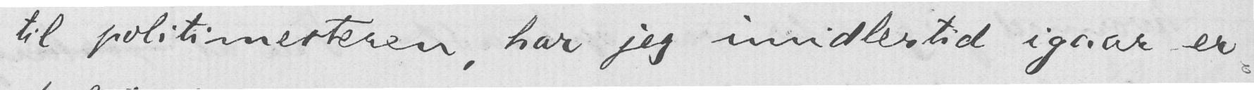til politimesteren, har jeg imidlertid igaar er-
